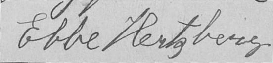Ebbe Hertzberg.
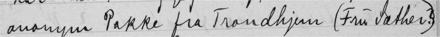anonym Pakke fra Trondhjem (Fru Sæther?)
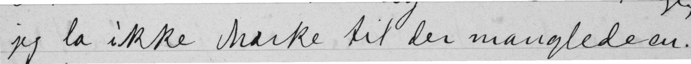jeg la ikke Mærke til der manglede en.
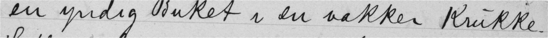en yndig Buket i en vakker Krukke.
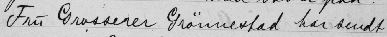Fru Grosserer Grønnestad har sendt
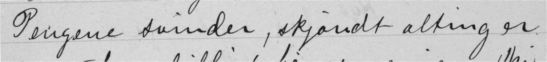Pengene svinder, skjøndt alting er
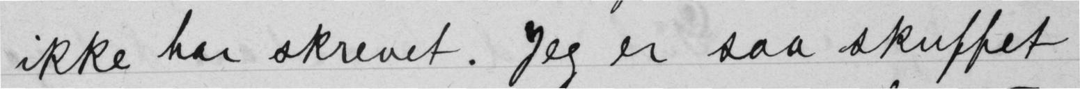ikke har skrevet. Jeg er saa skuffet
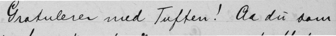Gratulerer med Tuften! Aa du som
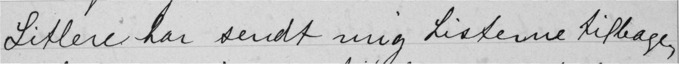Litlere har sendt mig Listerne tilbage,
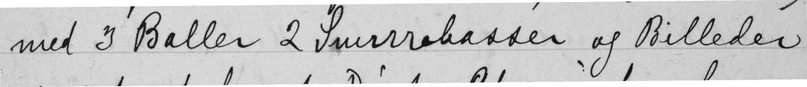med 3 Baller 2 snurrebasser og Billeder
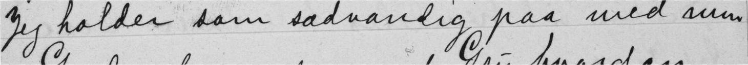Jeg holder som sædvandig paa med sum
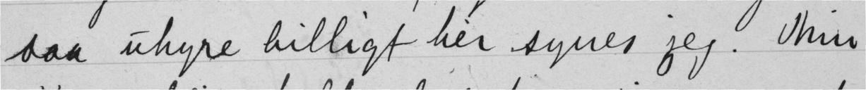saa uhyre billigt her synes jeg. Min
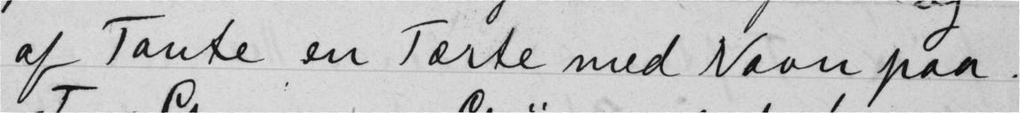af Tante en Tærte med Navn paa.
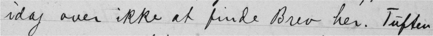idag over ikke at finde brev her. Tuften
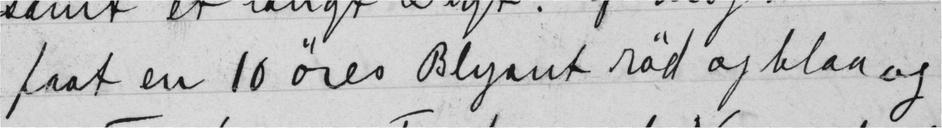faat en 10 øres Blyant rød og blaa og
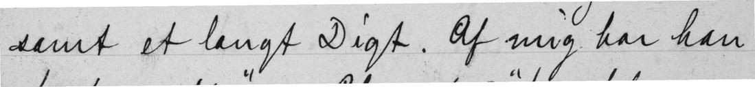samt et langt Digt. Af mig har han
